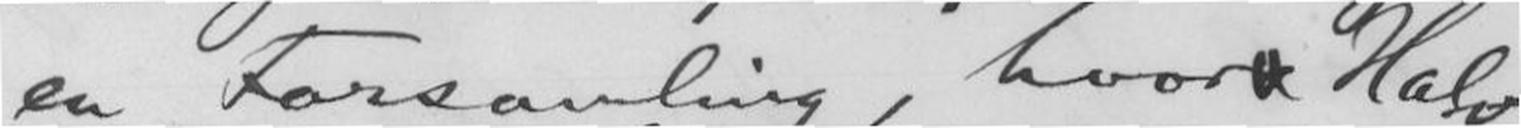en Forsamling, hvor Halv-
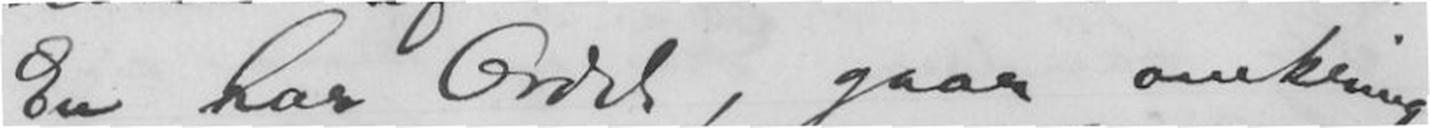En har Ordet, gaar omkring
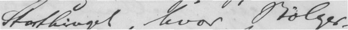Storthinget, hvor Bølger-
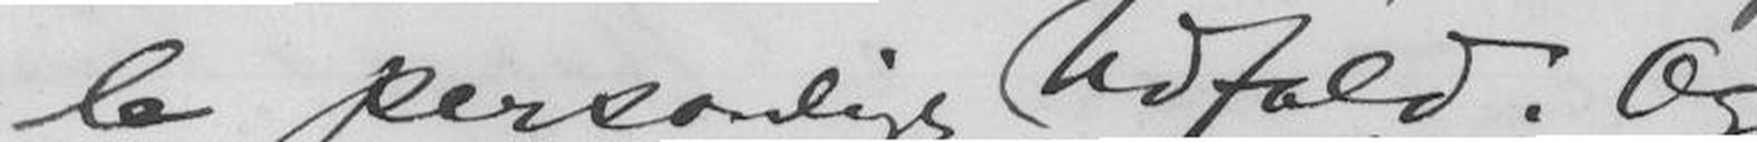le personlige Udfald. Og
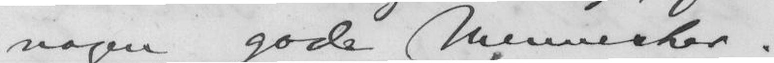nogen gode Mennesker!
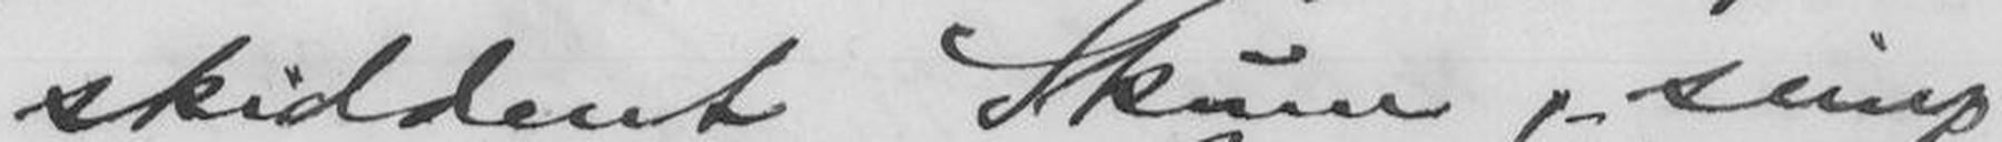skiddent Skum, simp-
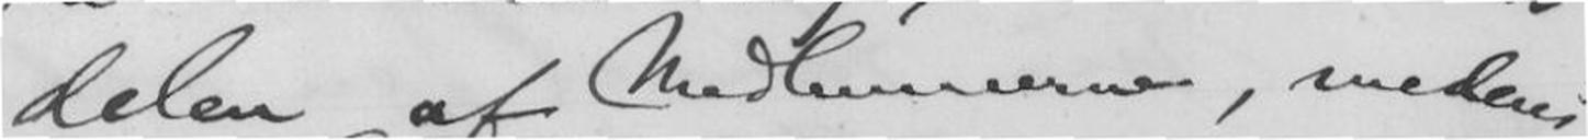delen af Medlemmerne, medens
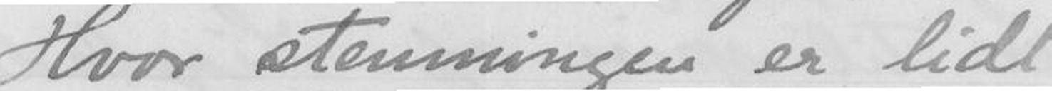Hvor stemningen er lidt
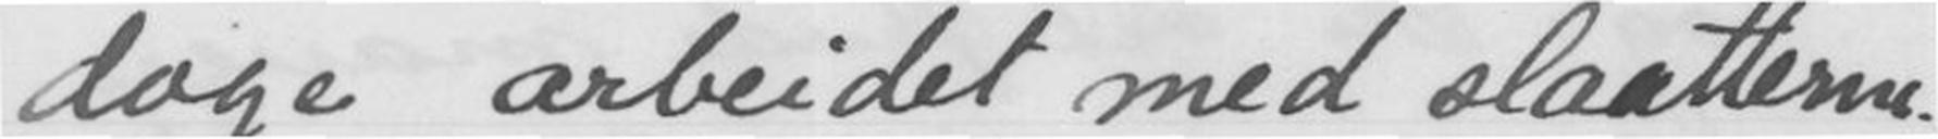dage arbeidet med slaatterne.
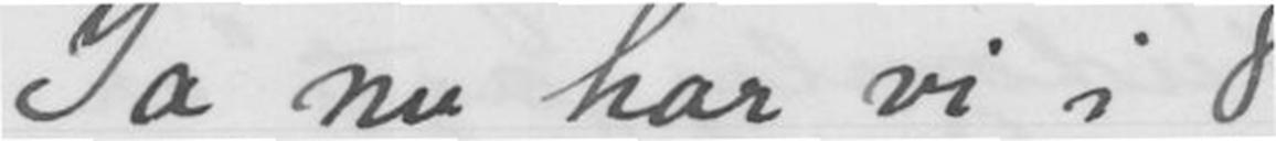Ja nu har vi i 8
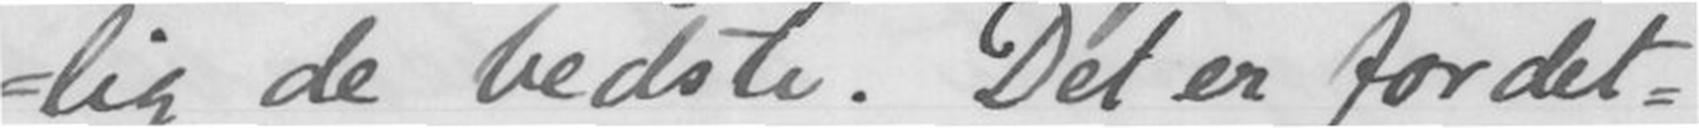lig de bedste. Det er fordet-
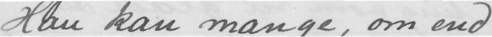Han kan mange, om end
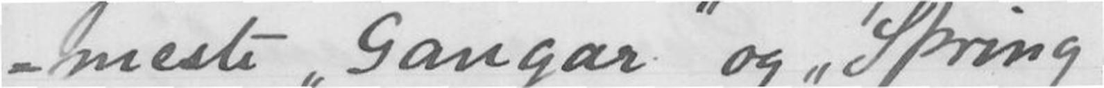meste Gangar og Spring-
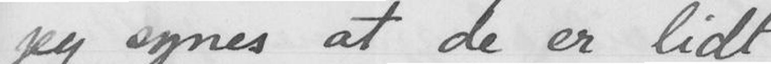jeg synes at de er lidt
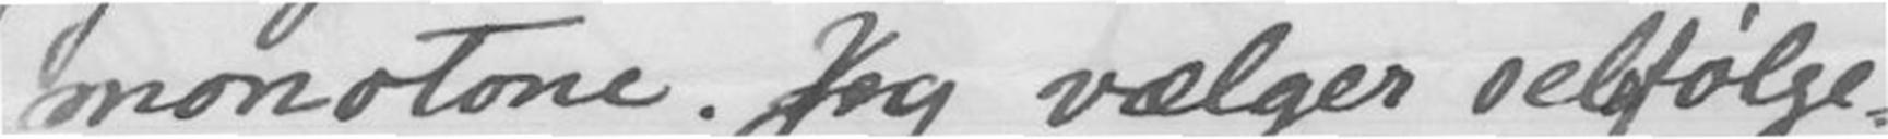monotone. Jeg vælger selvfølge-
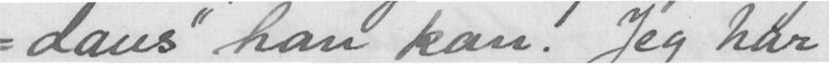dans han kan. Jeg har
